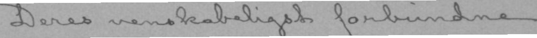Deres venskabeligst forbundne
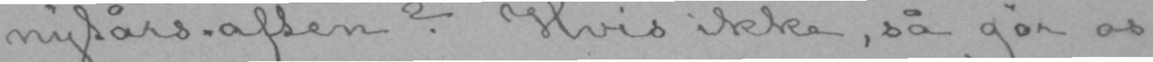nytårs-aften? Hvis ikke, så gør os
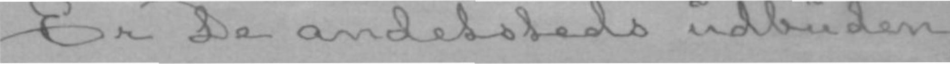Er De andetsteds udbuden
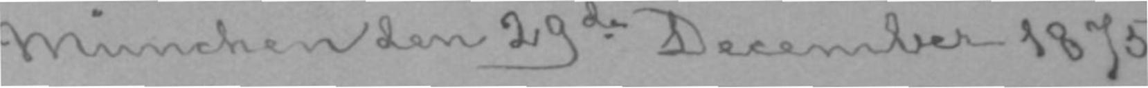München den 29de December 1875.
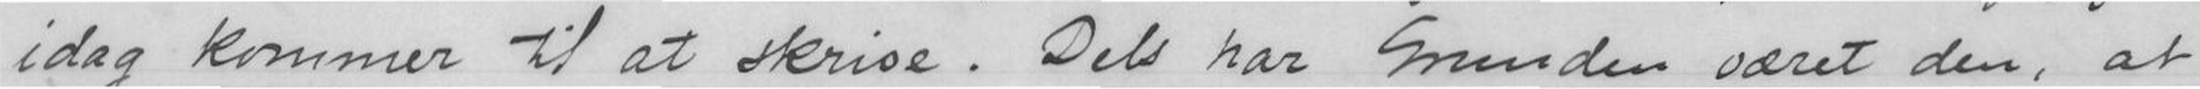idag kommer til at skrive. Dels har Grunden været den, at
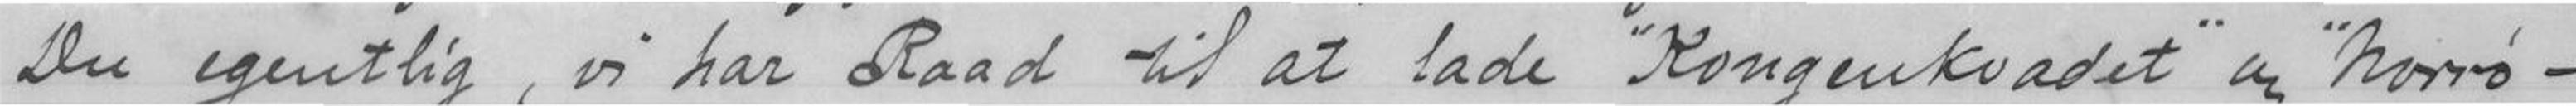Du egentlig, vi har Raad til at lade "Kongenkvadet" og "Norrø-
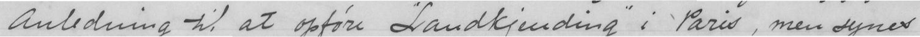Anledning til at opføre "Landkjending" i Paris, men synes
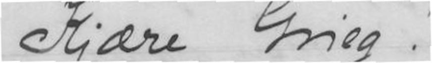Kjære Grieg!
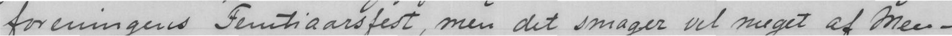foreningens Femtiaarsfest, men det smager vel meget af Men.-
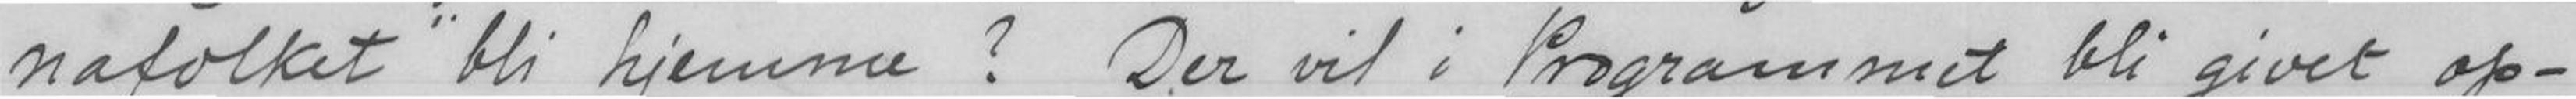nafolket" bli hjemme? Der vil i Programmet bli givet op-
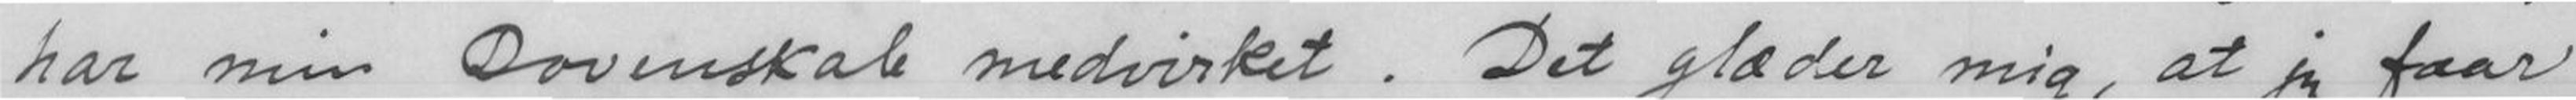har min Dovenskab medvirket. Det glæder mig, at jeg faar
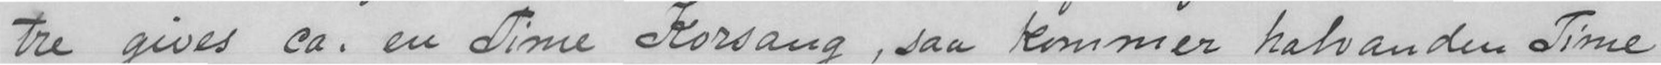tre gives ca. en Toime Korsang, saa kommer halvanden Time
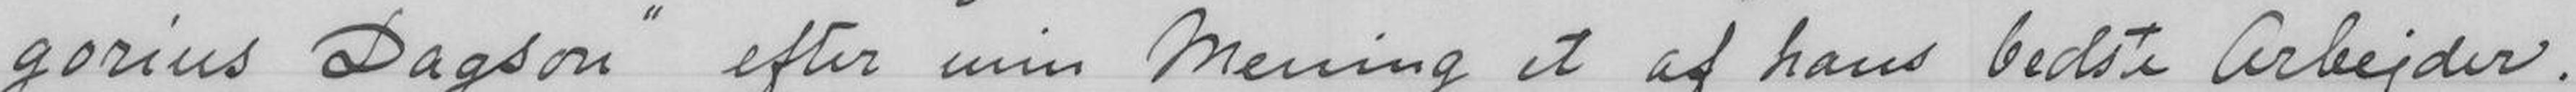gorius Dagson" efter min Mening et af hans bedste Arbejder.
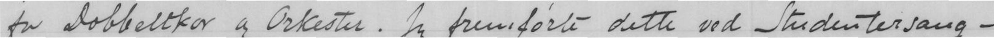for Dobbeltkor og Orkester. Jeg fremførte dette ved Studentersang-
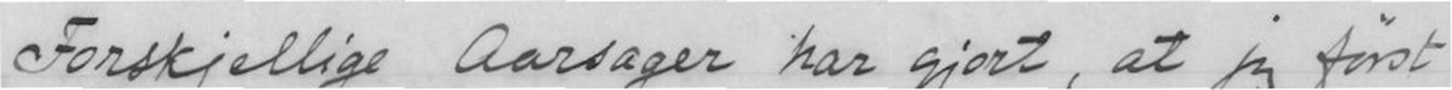Forskjellige Aarsager har gjort, at jeg først
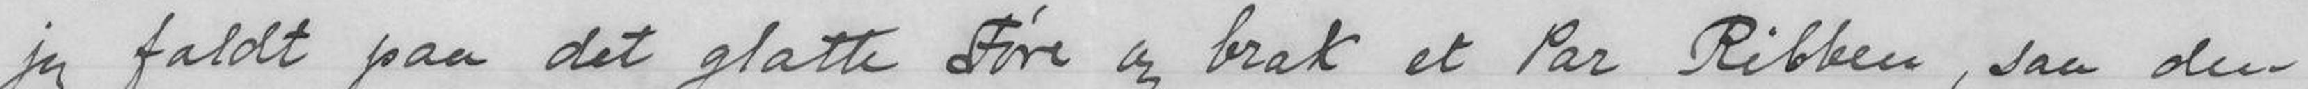jeg faldt paa det glatte Føre og brak et Par Ribben, saa den
